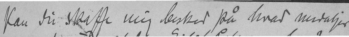Kan du skaffe mig besked på hvad medaljer
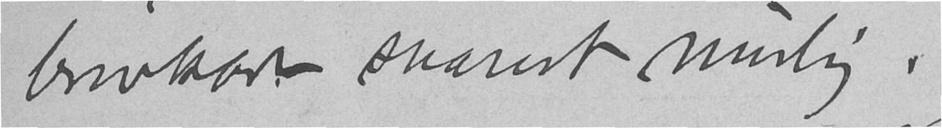brevkort snarest mulig.
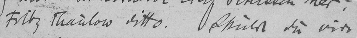Fritz Thaulow ditto. Skulde du vide
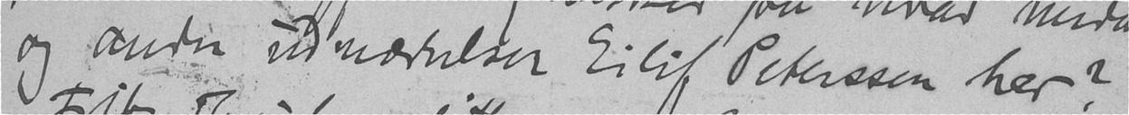og andre udmærkelser Eilif Peterssen har?
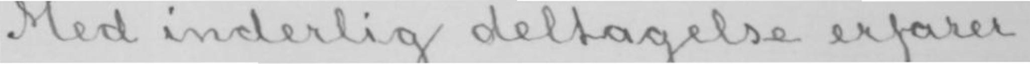Med inderlig deltagelse erfarer
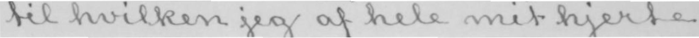til hvilken jeg af hele mit hjerte
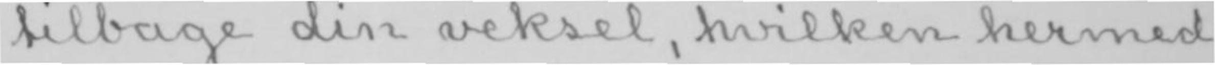tilbage din veksel, hvilken hermed
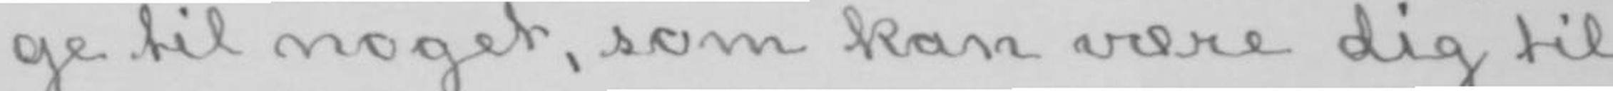ge til noget, som kan være dig til
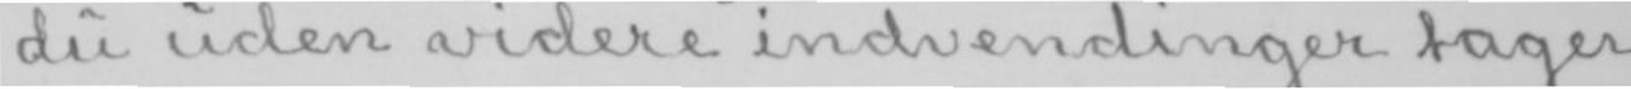du uden videre indvendinger tager
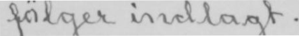følger indlagt.
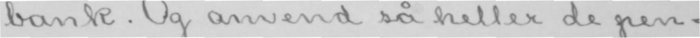bank. Og anvend så heller de pen-
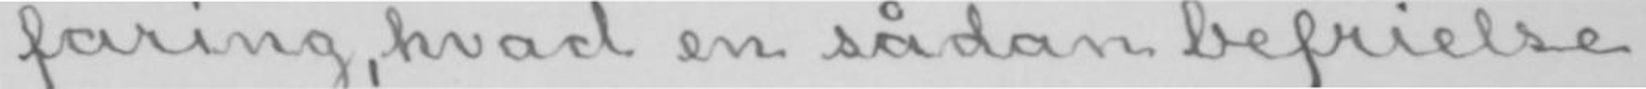faring, hvad en sådan befrielse
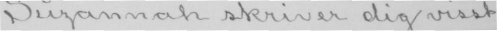Suzannah skriver dig visst
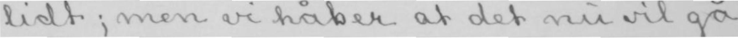lidt; men vi håber at det nu vil gå
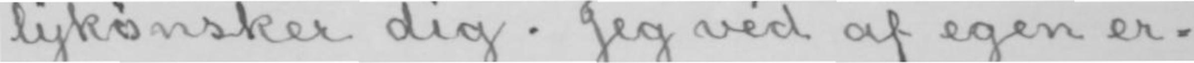lykønsker dig. Jeg véd af egen er-
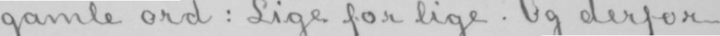gamle ord: Lige for lige. Og derfor
